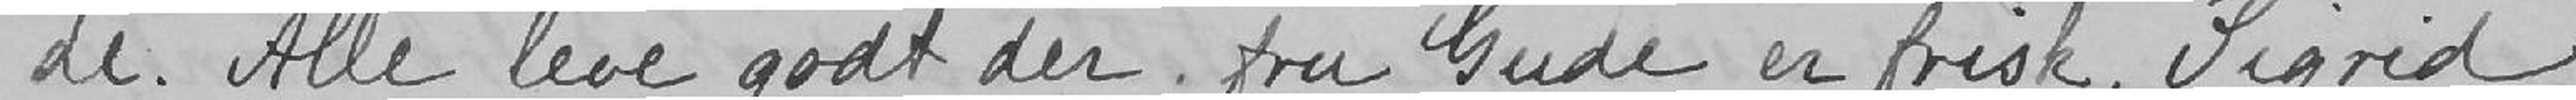Alle leve godt der; fru Gude er frisk, Sigrid
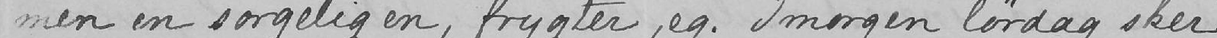men en sørgelig en, frygter jeg. Imorgen lørdag sker
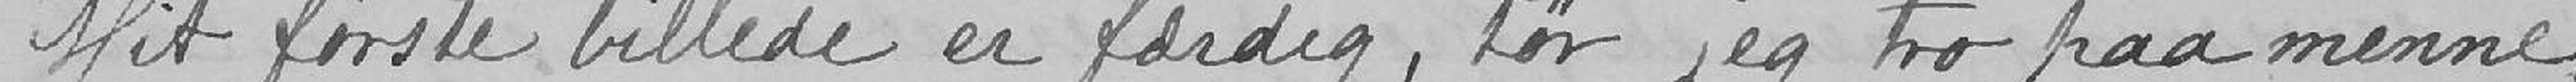Mit første billede er færdig, tør jeg tro paa menne
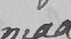maa
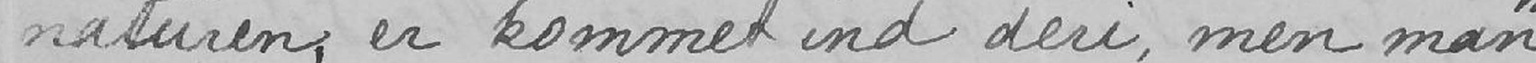naturen, er kommet ind deri, men man
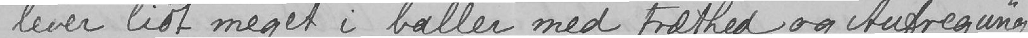lever lidt meget i baller med træthed og «Aufregung»
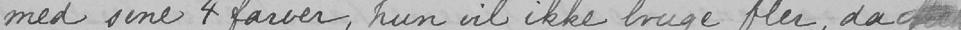med sine 4 farver, hun vil ikke bruge fler,
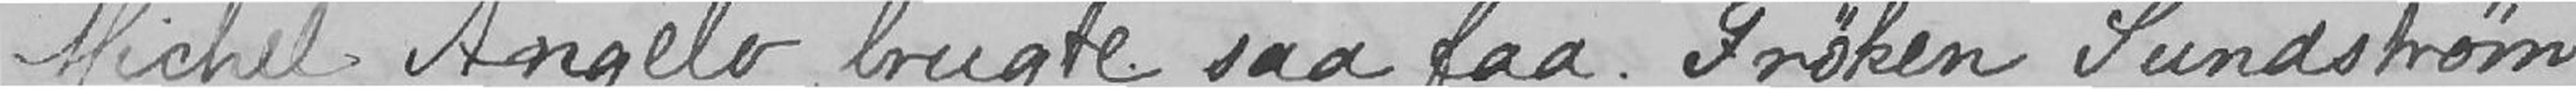da Michel Angelo brugte saa faa. Frøken Sundstrøm
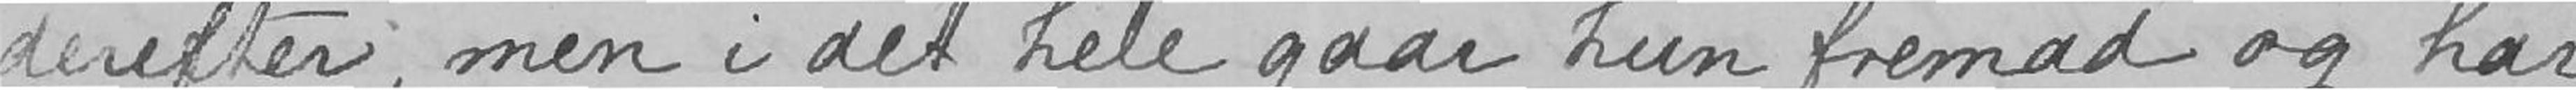derefter, men i det hele gaar hun fremad og har
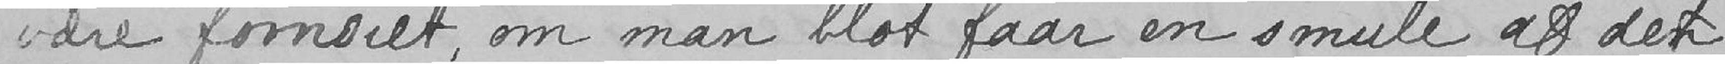være fornøiet, om man blot faar en smule af det
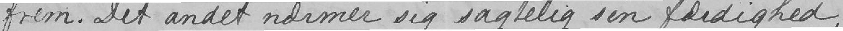frem. Det andet nærmer sig sagtelig sin færdighed,
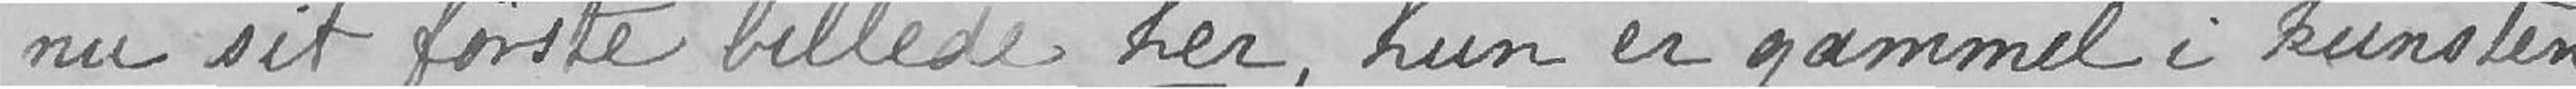nu sit første billede her, hun er gammel i kunsten.
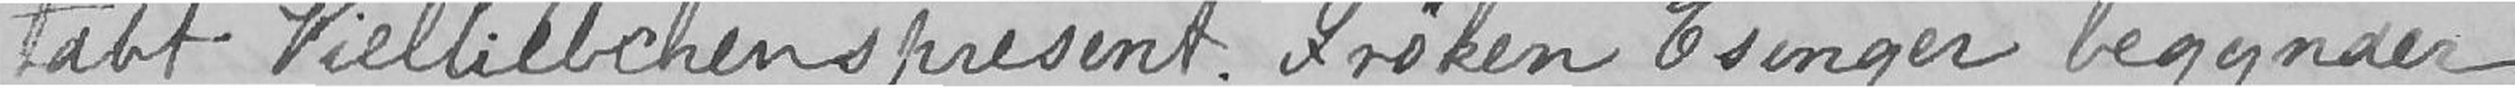tabt Vielliebchenspresent. Frøken Esinger begynder
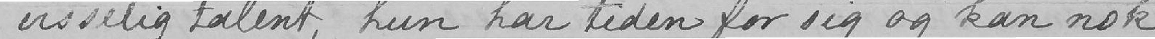visselig talent, hun har tiden for sig og kan nok
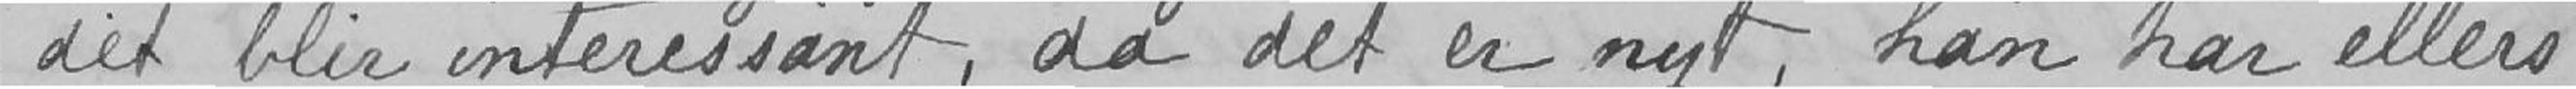det blir interessant, da det er nyt, han har ellers
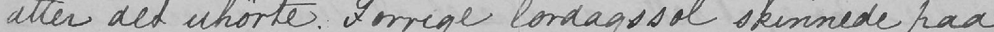atter det uhørte. Forrige lørdagssol skinnede paa
